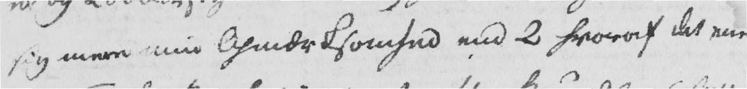sig mere min Opmærksomhed end 2 hvoraf det ene
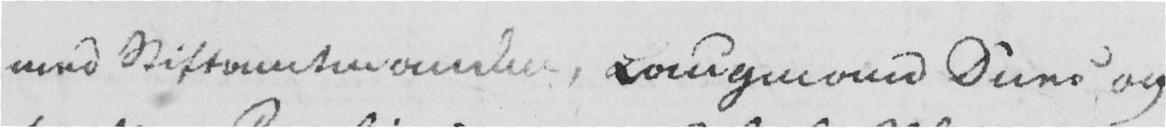med Stiftamtmanden, Laugmand Duns og
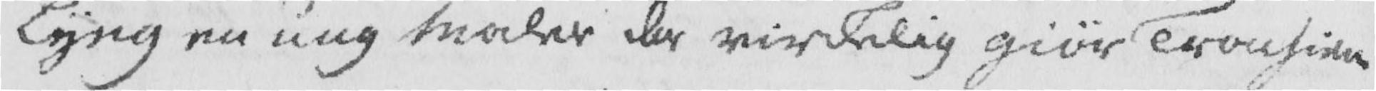Lyng en ung Maler der virkelig giør Tronhiem
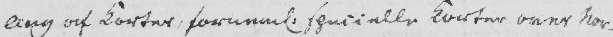ling af Korter, forneml. specielle Korter over Nor-
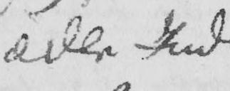ædle Ind
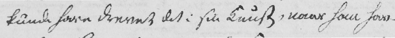kunde have drevet det i sin Kunst, naar han hav-
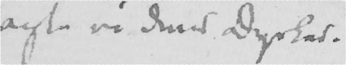agte vi dend Dyrked.
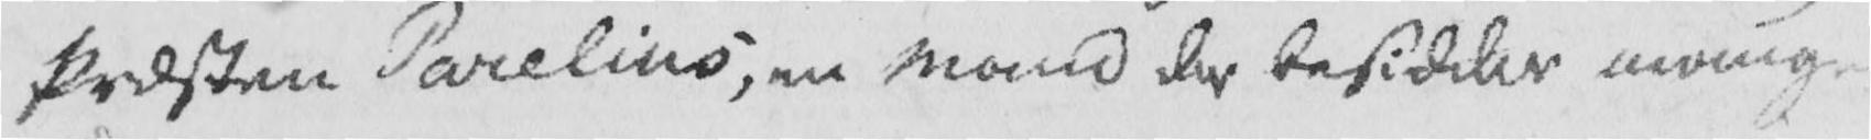Præsten Parelius, en Mand der besidder mange
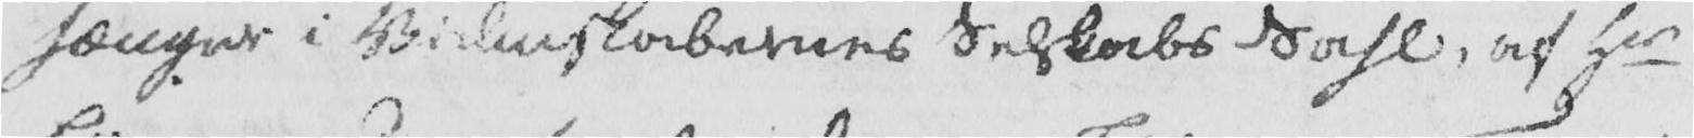hænger i Videnskabernes Selskabs Sahl, af Hr
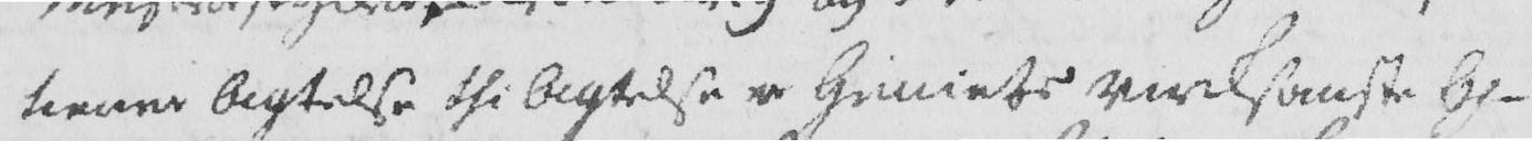tiener Agtelse thi Agtelse er Geniets virksomste Op-
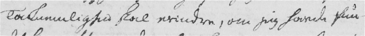Taknemlighed skal erindre, om jeg havde fun-
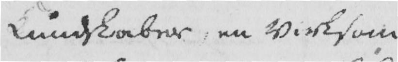Kundskaber, er Virksom
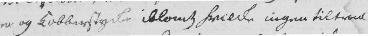er og Kobberstycke iblant hvilke ingen tiltrak
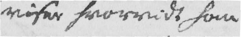viser hvorvidt han
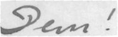Dem!
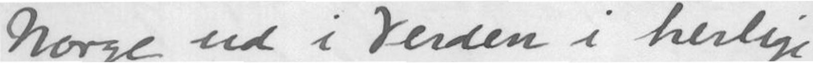Norge ud i Verden i herlige
